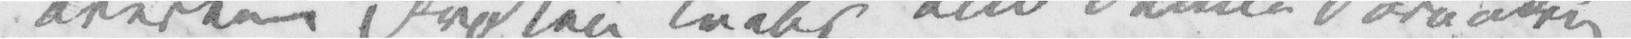avertere Frøken Krebs om denne Forandring[.]
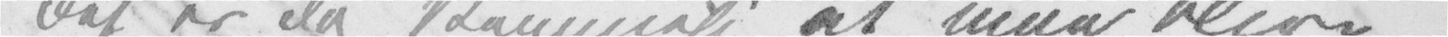Det er dog skammelig at man blive
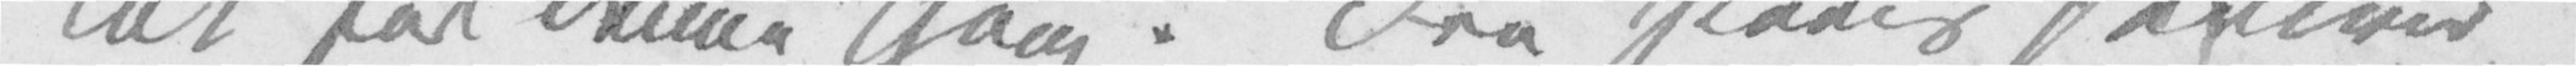Tak for denne Gang. Fra Paris skriver
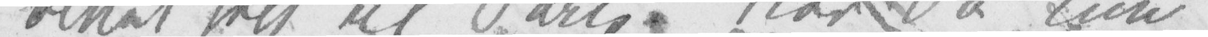billett helt til Paris. Når De kun
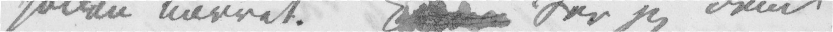sådan narret. K<gap /></del> Ser jeg Dem
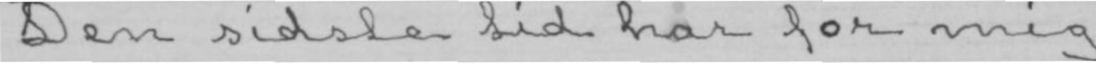Den sidste tid har for mig
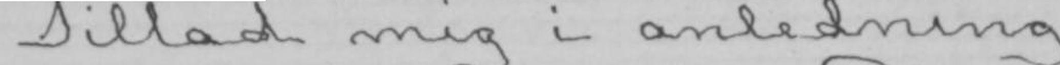Tillad mig i anledning
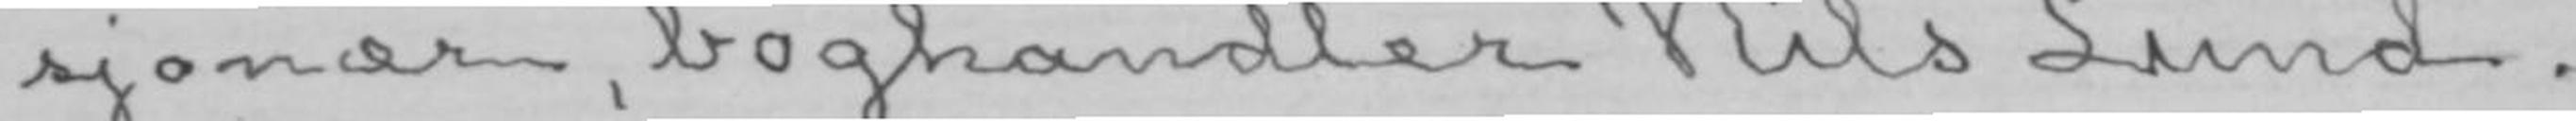sjonær, boghandler Nils Lund.
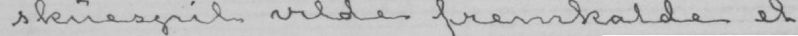skuespil vilde fremkalde et
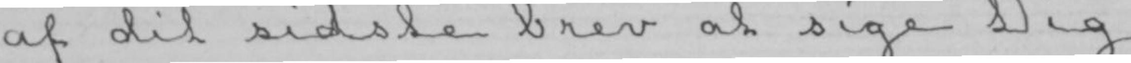af dit sidste brev at sige Dig
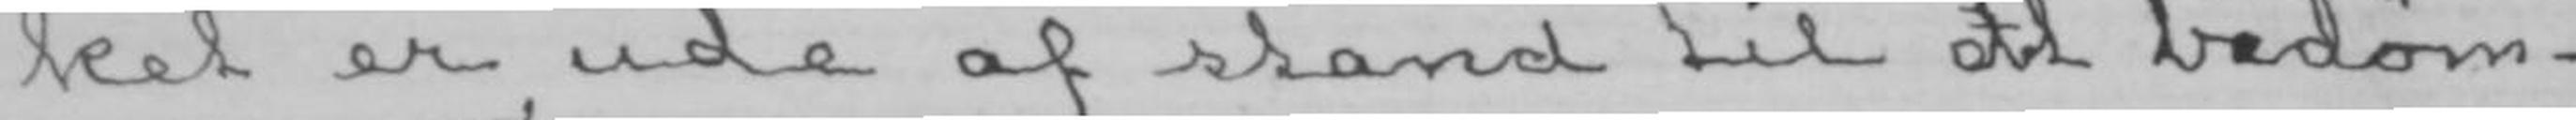ket er ude af stand til dat bedøm-
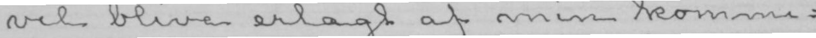vil blive erlagt af min kommi-
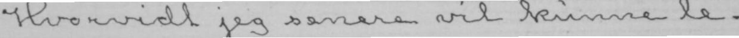Hvorvidt jeg senere vil kunne le-
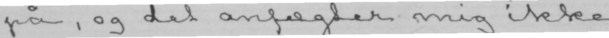på, og det anfægter mig ikke
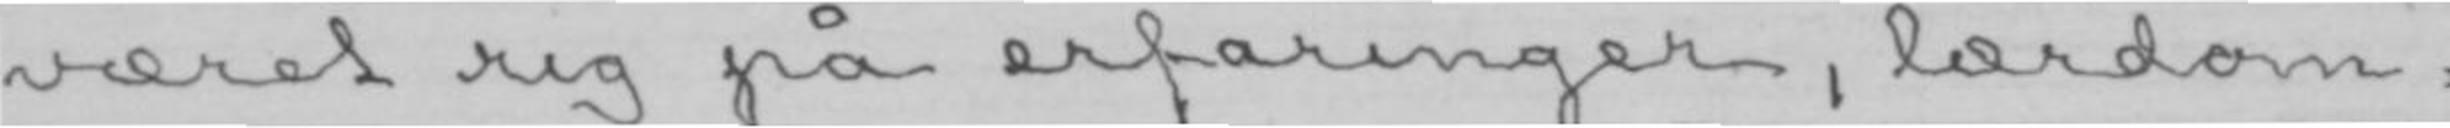været rig på erfaringer, lærdom-
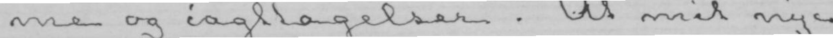me og iagttagelser. At mit nye
